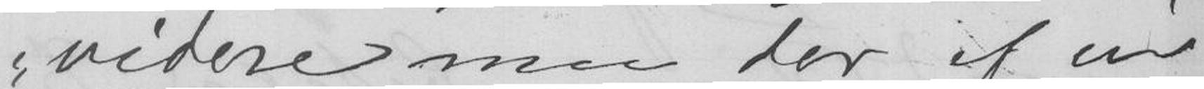videre maa der af en
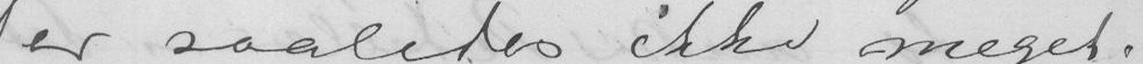er saaledes ikke meget.
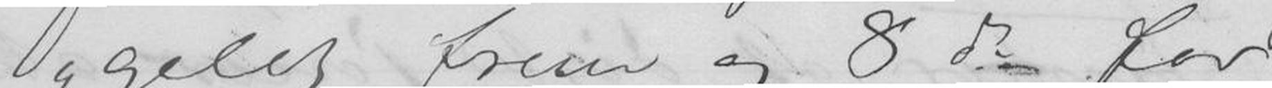gelet frem og 8 do for
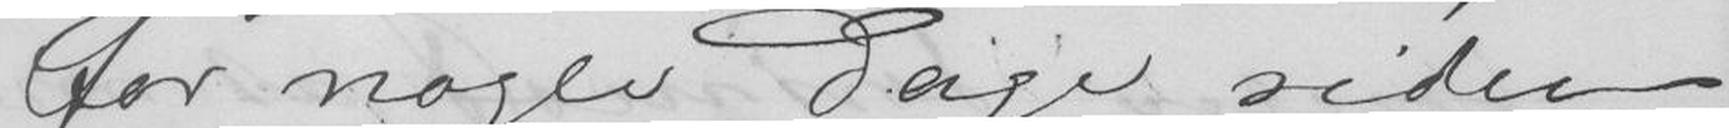for nogle Dage siden
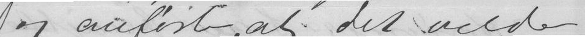og anførte at det vilde
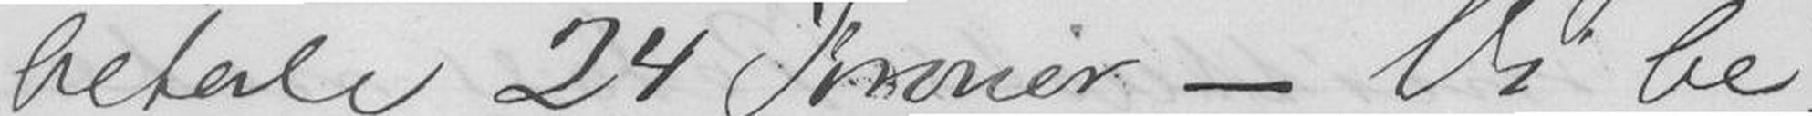betale 24 Kroner - Vi be-
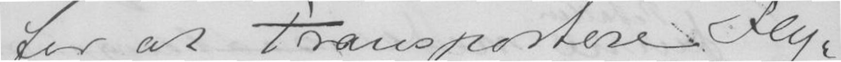for at Transportere Fly-
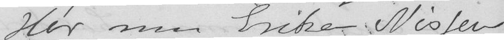Her maa Erika Nissen
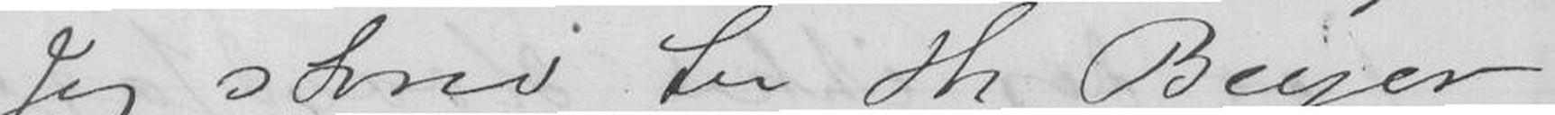Jeg skrev til Hr Beyer
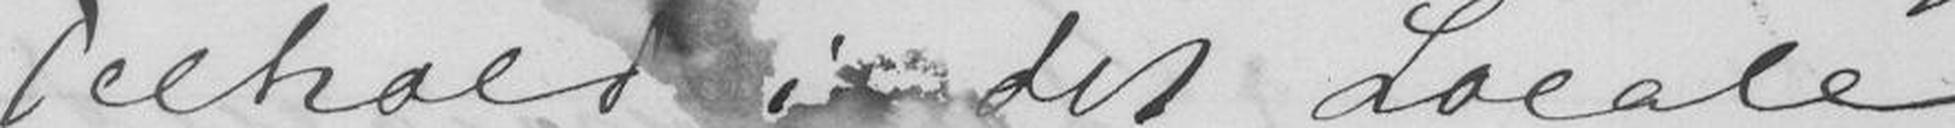Tilhold i det Locale
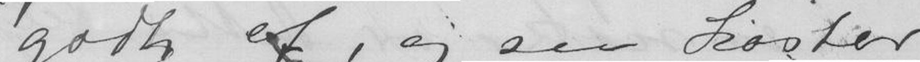godt af, og saa koster
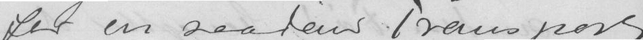for en saadan Transport
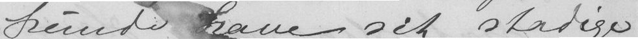kunde have sit stadige
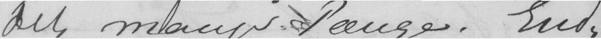det mange Pænge. End-
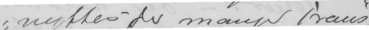nyttes De mange Trans
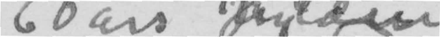60 års Hylden
